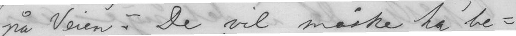på Veien. De vil måske ha be-
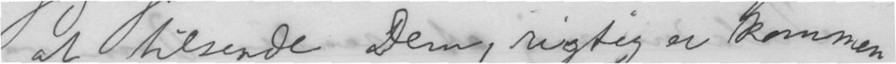at tilsende Dem, rigtig er kommen
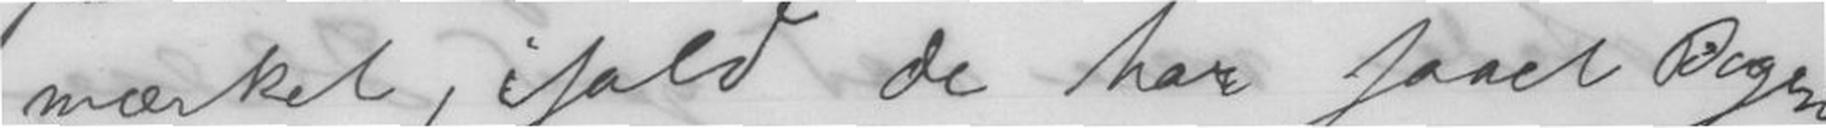mærket, ifald de har faaet Bogen,
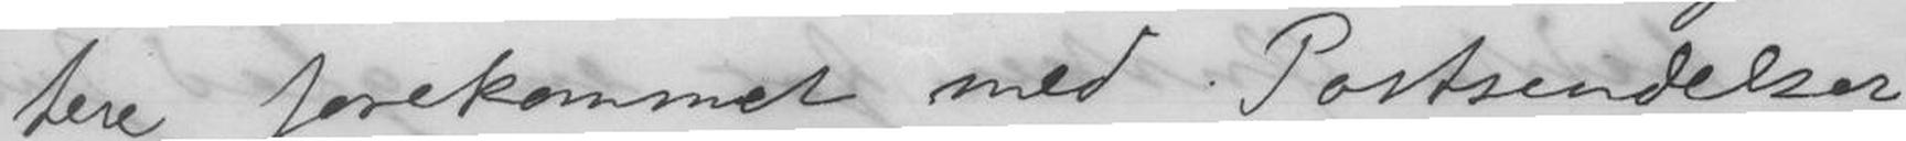tere forekommet med Postsendelser
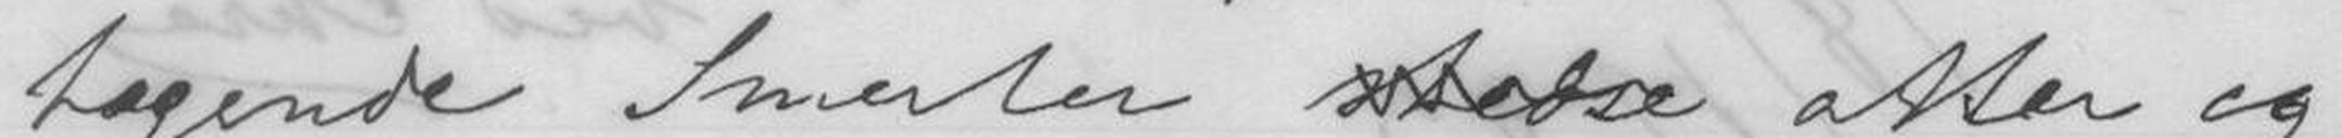tagende Smerter stedse atter og
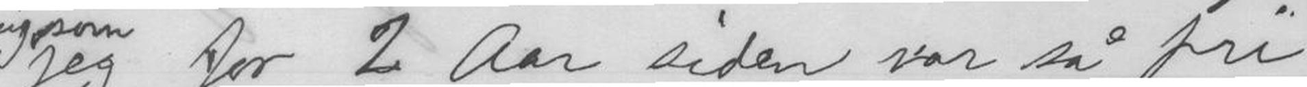jeg for 2 Aar siden var så fri
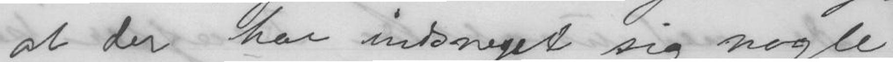at der har indsneget sig nogle
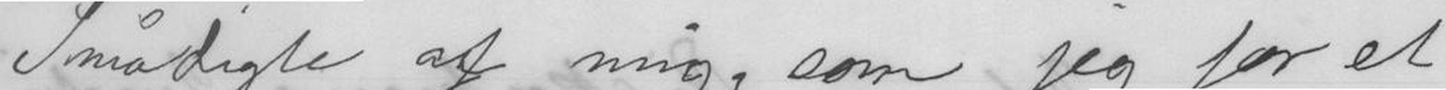Smådigte af mig, som jeg for et
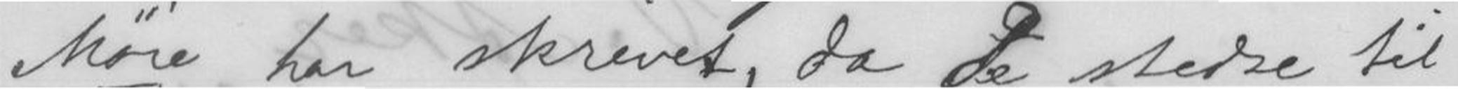Møie har skrevet, da De stedse til-
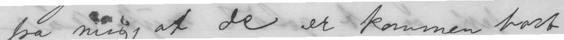fra mig, at de er kommen bort
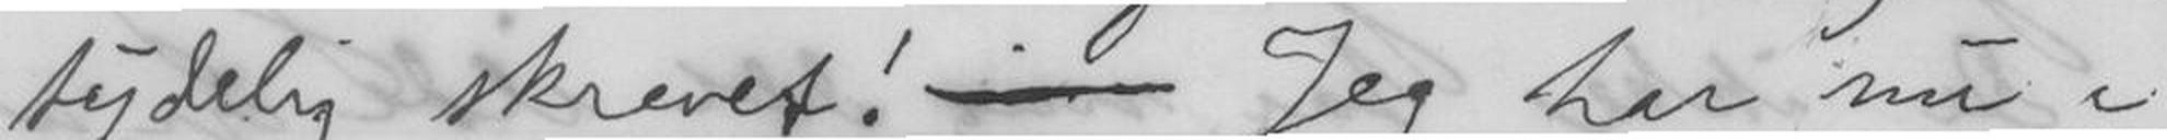tydelig skrevet! - Jeg har nu i
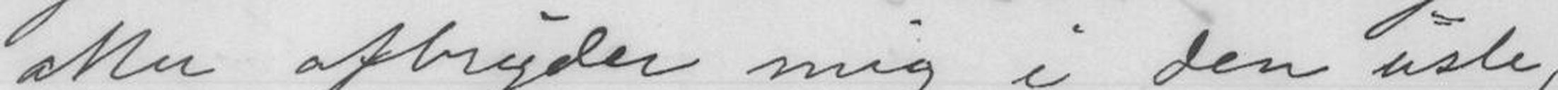atter afbryder mig i den usle,
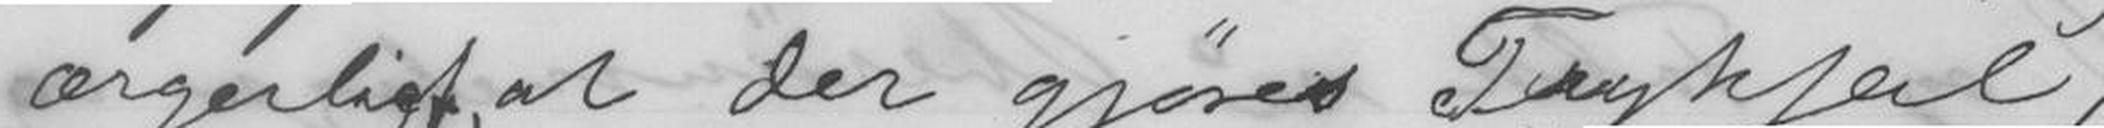ærgerligt, at der gjøres Trykfeil,
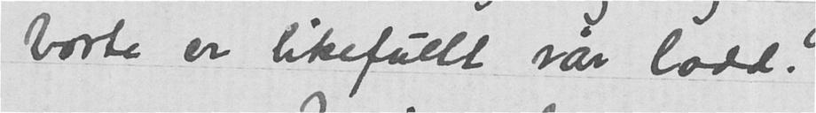borte er likefullt vår lodd.
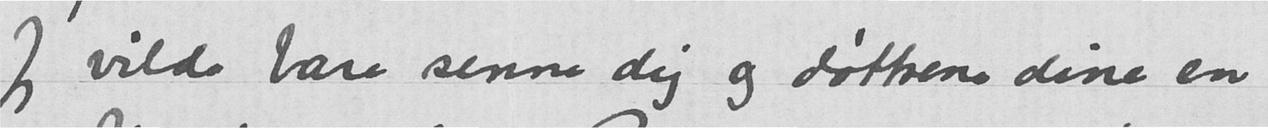Jeg vilde bare senne dig og døttrene dine en
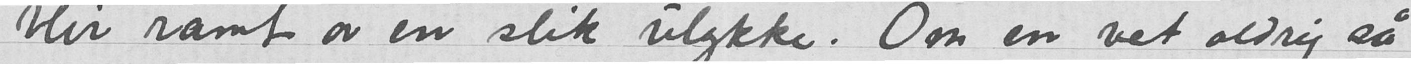blir ramt av en slik ulykke. Om en vet aldrig så
